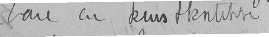bare en kunstkritiker
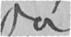da
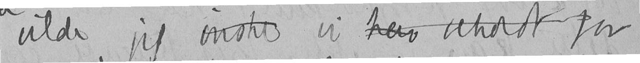vilde jeg ønske vi hav beholdt for
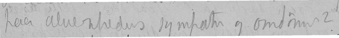paa almenhedens sympathi og omdømme?
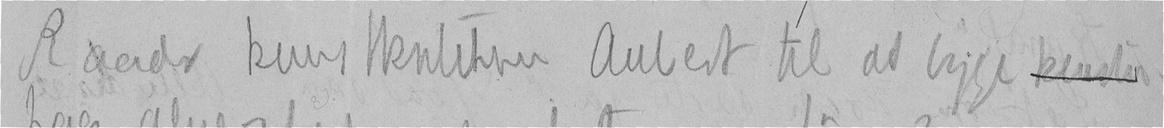Raader kunstkritikeren Aubert til at bygge kunde
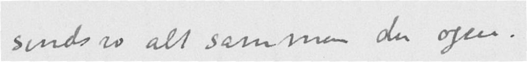sindsro alt sammen du ogsaa.
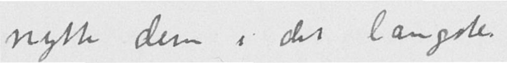nytte dem i det længste.
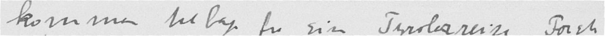kommen tilbage fra sin Tyrolerreise. Første
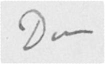Din
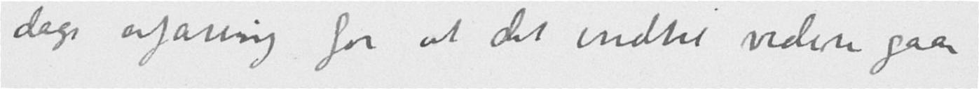dags erfaring for at det indtil videre gaar
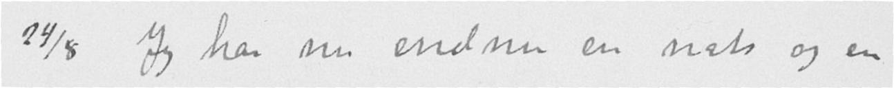24/8 Jeg har nu endnu en nats og en
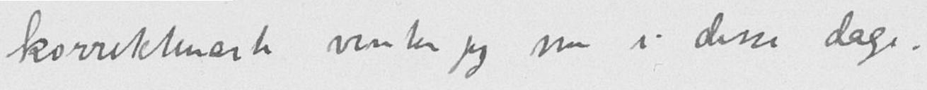korrekturark venter jeg nu i disse dage.
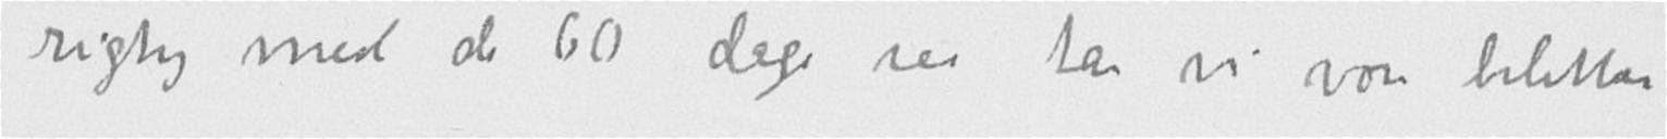rigtig med de 60 dage saa tar vi vore biletter
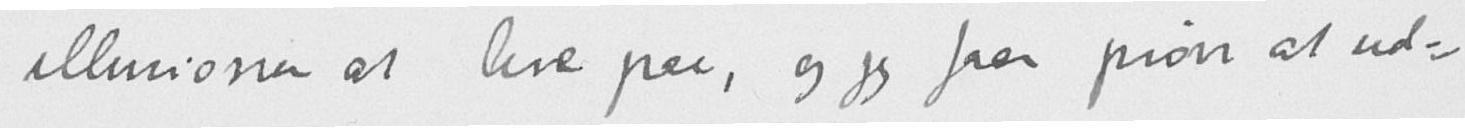illusioner at leve paa, og jeg faar prøve at ud-
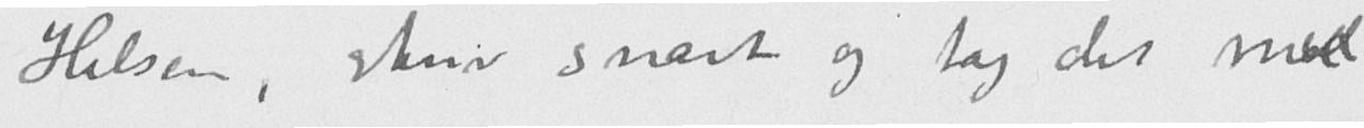Hilsen, skriv snart og tag det med
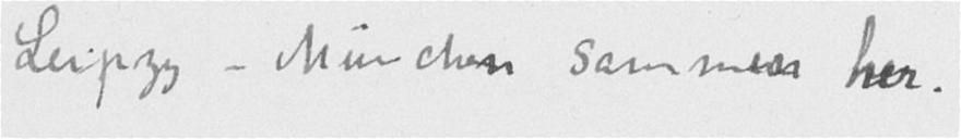Leipzig-München sammen her.
